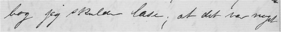bog jeg skulde læse; at det var noget
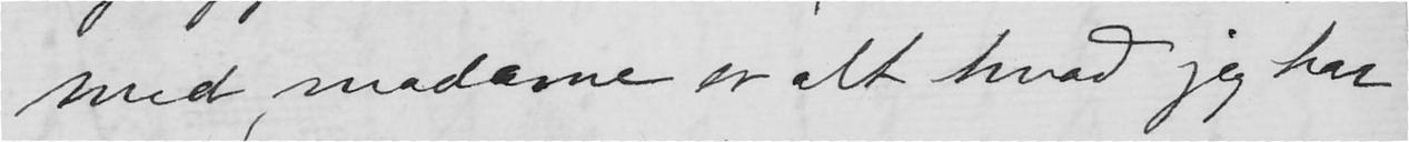med madame er alt hvad jeg har
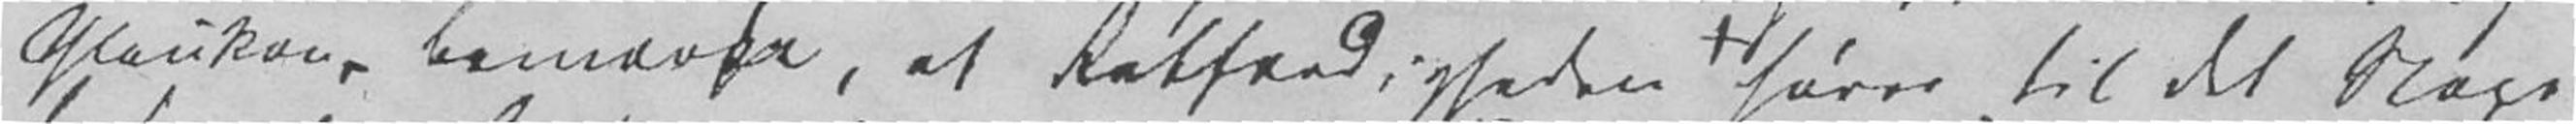Glaukon bemærke, at Retfærdigheden hører til det Slags
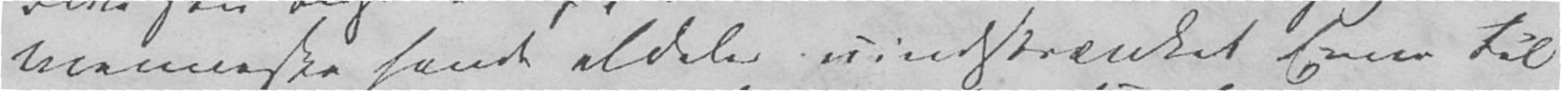Menneske havde aldeles uindskrænket Evne til
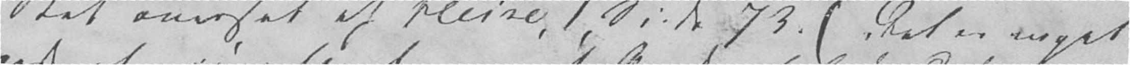Stat oversat af Heine, I, Side 73. ( Det er noget
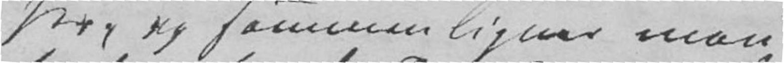ser, og sammenligner man
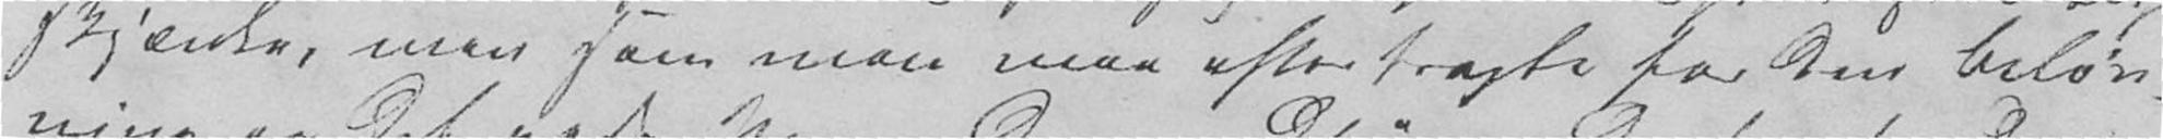skjænke, men som man maa eftertragte for dens Beløn-
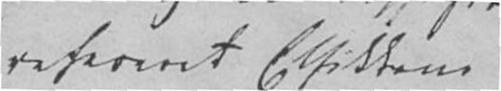reserveret Ethikens
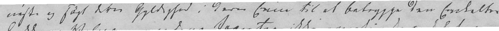neske og søgt deres Gyldighed i deres Evne til at betrygge den Enkeltes
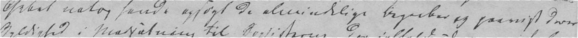Gebet netop havde opsøgt de almindelige Begreber og paavist deres
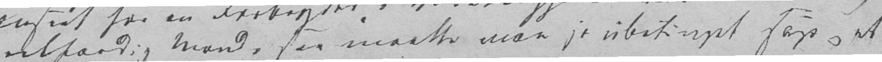retfærdig Mand, saa maatte man jo ubetinget sige, at
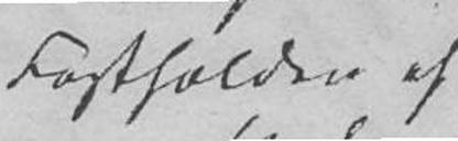Fastholden af
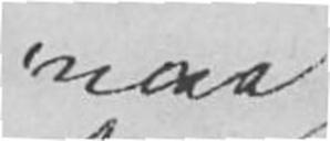maa
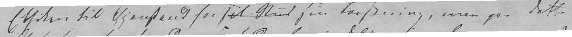Ethiken til Gjenstand for sit Stud sin Forskning, men paa dette
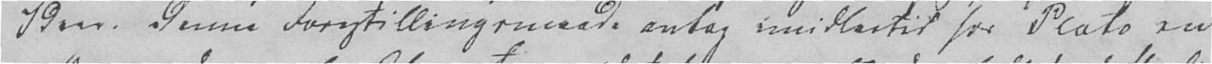Ideer. Denne Forestillingsmaade antog imidlertid hos Plato en
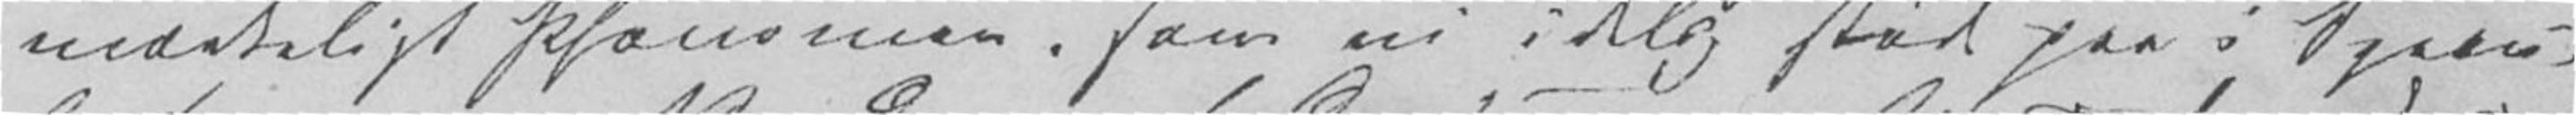mærkeligt Phænomen, som vi idelig støde paa i Specu-
